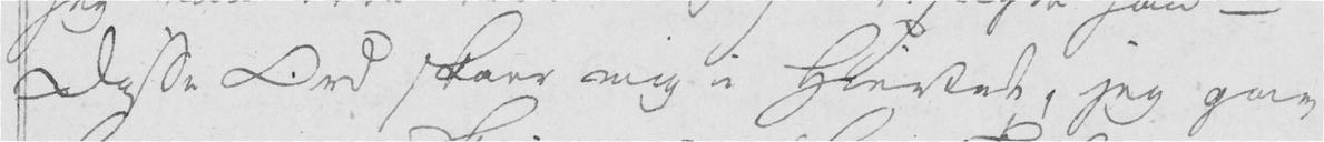Disse Ord skaer mig i Hiertet, jeg gav
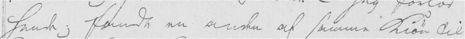hende; fandt en anden af samme Kiøn til
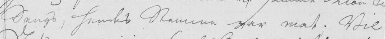Sengs, hendes Stemme var mat. Vil
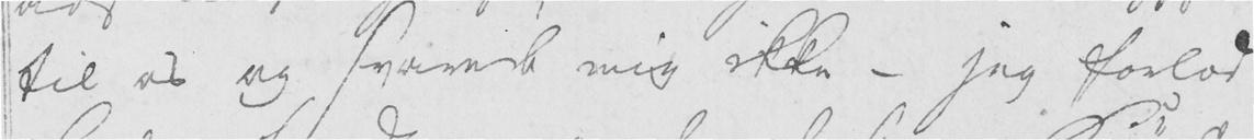til os og svarede mig ikke - jeg forlod
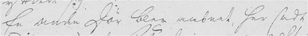En anden Dør blev aabnet, her sadt
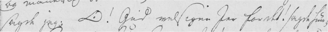sagde jeg. O! Gud velsigne Jer for det! sagde hun;
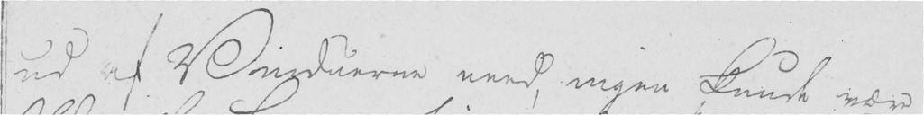ud af Vinduerne need, ingen kunde være
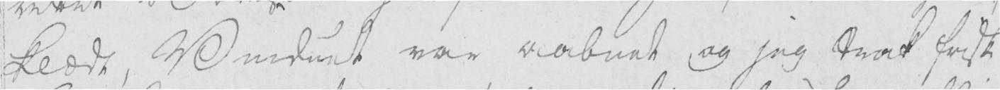klædt, Vinduet var aabnet og jeg trak frisk
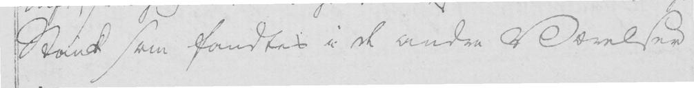Stank som fandtes i de andre Værelser
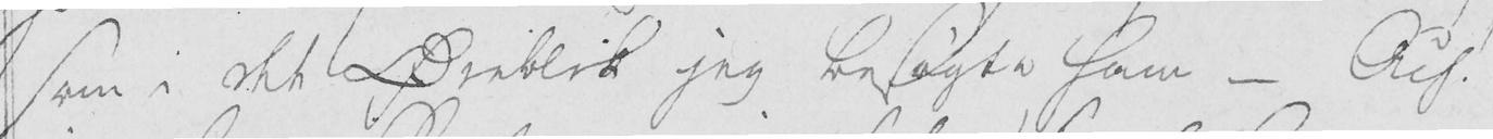som i det Øieblik jeg besøgte ham - Auf!
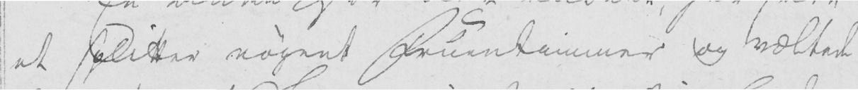et splitter nøgent Fruentimmer og væltede
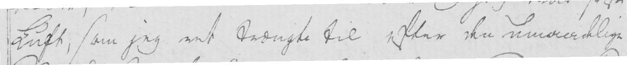Luft, som jeg ret trængte til efter den umaadelige
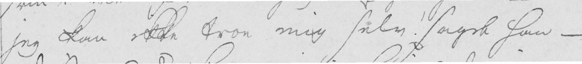jeg kan ikke troe mig selv! sagde han -
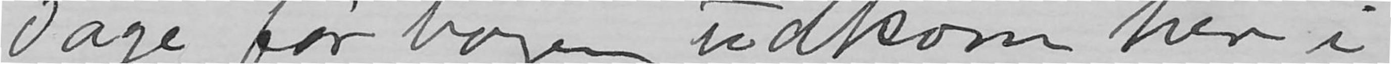dage før bogen udkom her i
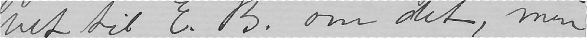vet til E.B. om det, men
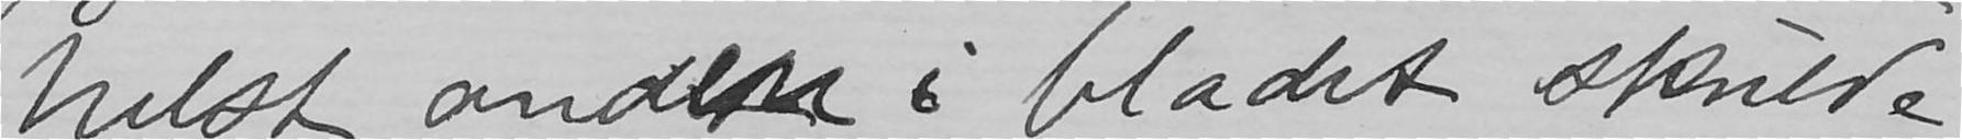helst anden i bladet skulde
